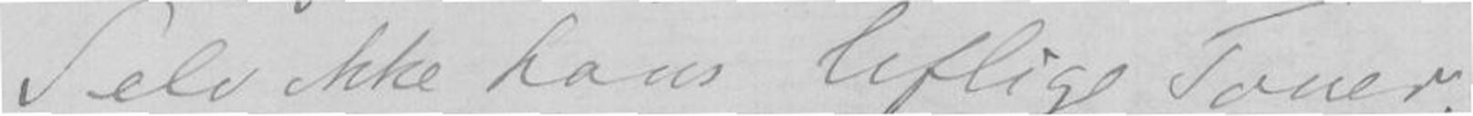Selv ikke hans liflige Toner.
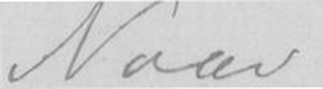Naar
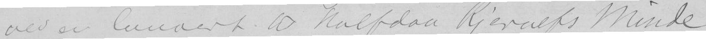ved en Concert til Halfdan Kjerulfs Minde.
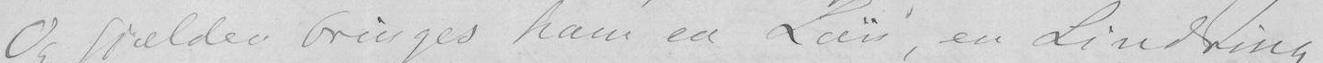Og sjælden bringes ham en Løn, en Lindring
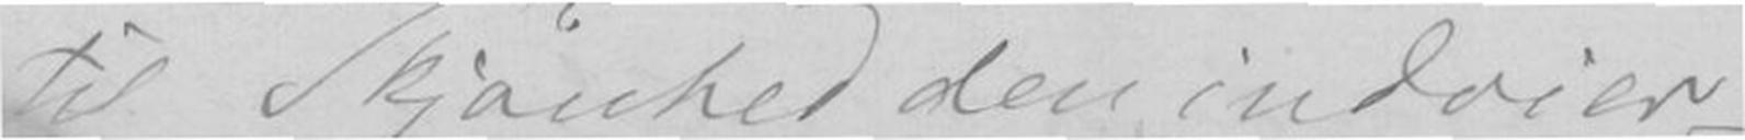til Skjønhed den indvier -
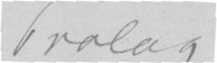Prolog
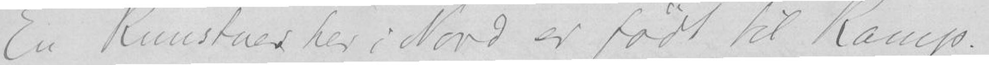En Kunstner her i Nord er født til Kamp.
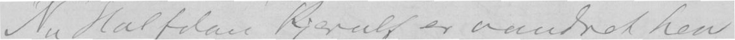"Nu Halfdan Kjerulf er vandret hen
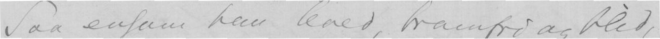Saa ensom han kved, bramfri og blid,
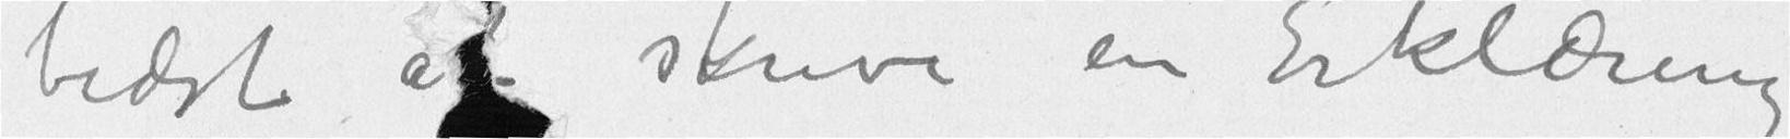bedst at skrive en Erklæring
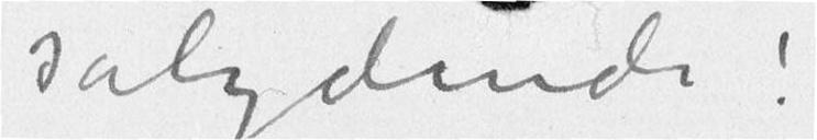sålydende:
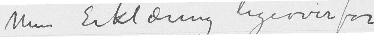Min Erklæring ligeoverfor
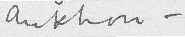Auktion –
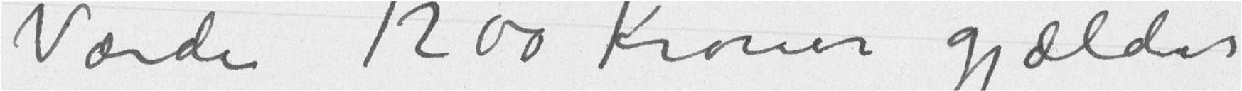Værdi 1200 Kroner gjælder
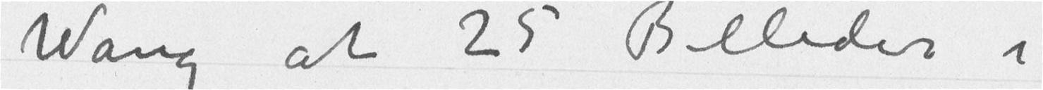Wang at 25 Billeder i
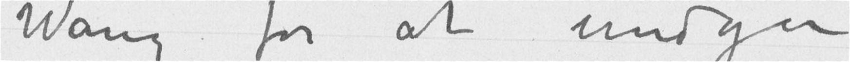Wang for at undgå
